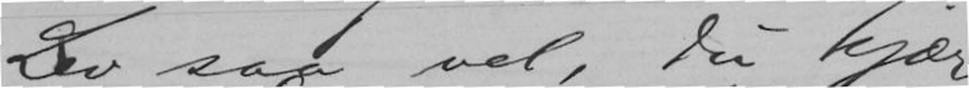Lev saa vel, Du kjære
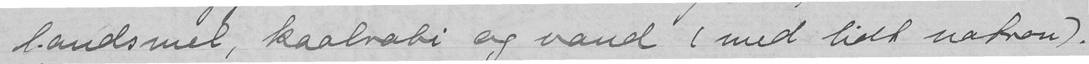landsmel, kaalrabi og vand (med lidt natron).
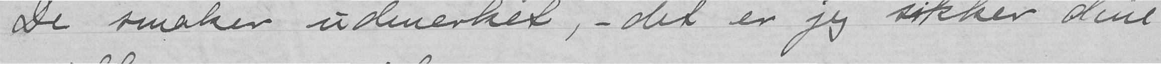De smaker udmerket, - det er jeg sikker dine
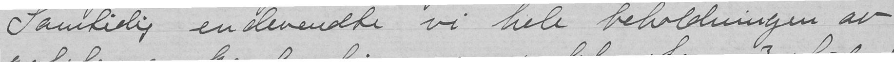Samtidig endevendte vi hele beholdningen av
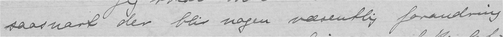saasnart der bliv nogen væsentlig forandring
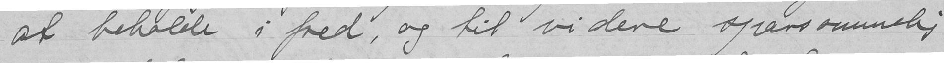at beholde i fred, og til videre sparsommelig
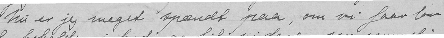Nu er jeg meget spendt paa, om vi faar lov
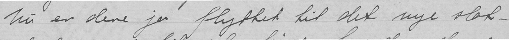Nu er dere jor flyttet til det nye slot-
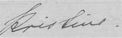Kristine.
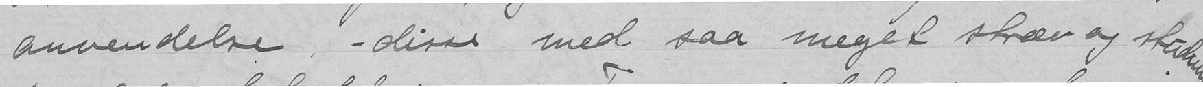anvendelse -disse med saa meget stræv og studium
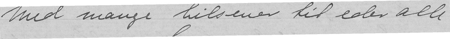Med mange tilsener til eder alle
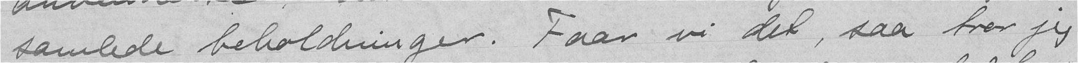samlede beholdninger. Faar vi det, saa tror jeg
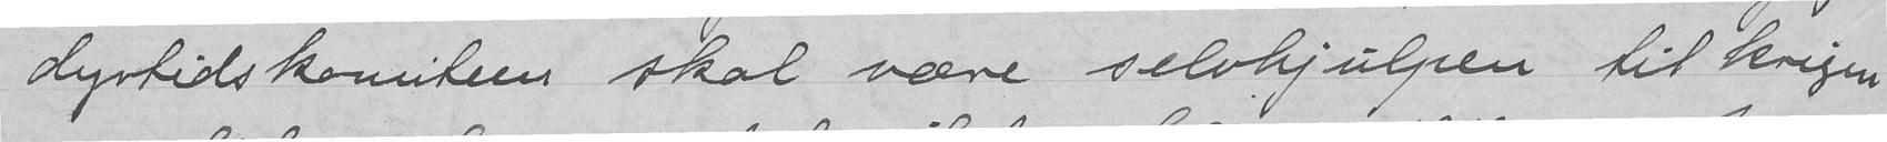dyrtidskomiteen skal være selvhjulpen til krigen
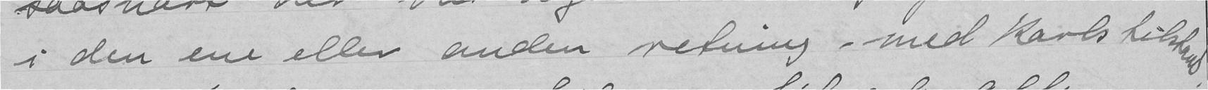i den ene eller anden retning med Karls tilstand
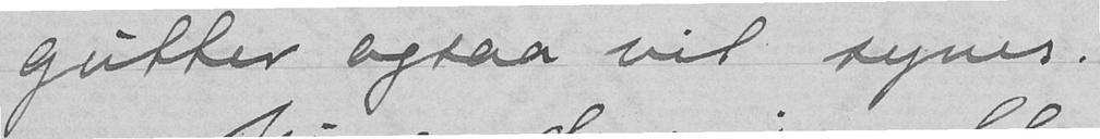gutter ogsaa vil synes.
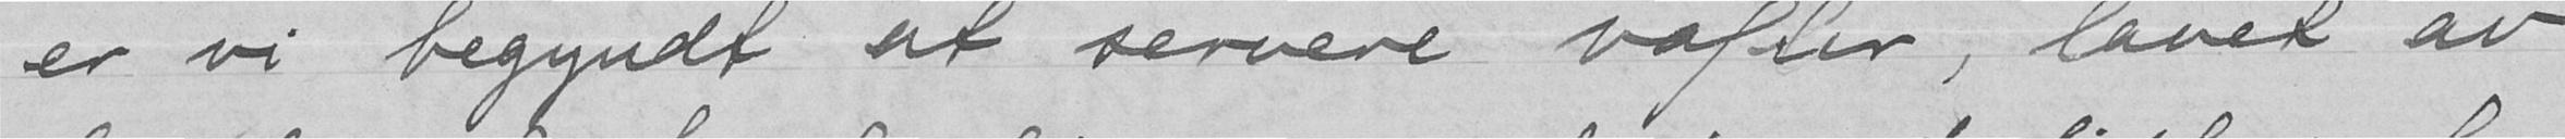er vi begyndt at servere vafler, lavet av
